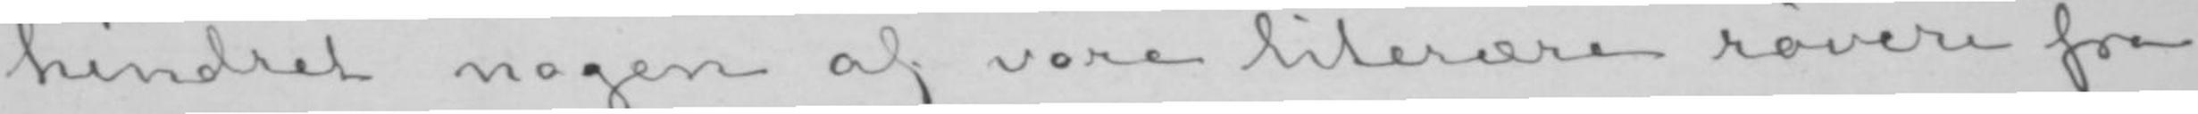hindret nogen af vore literære røvere fra
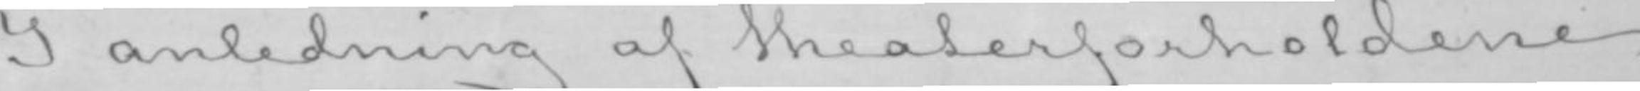I anledning af theaterforholdene
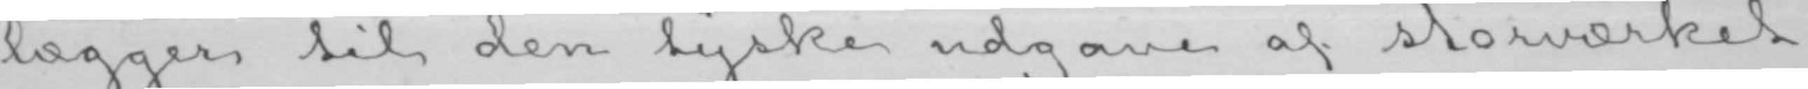lægger til den tyske udgave af storværket
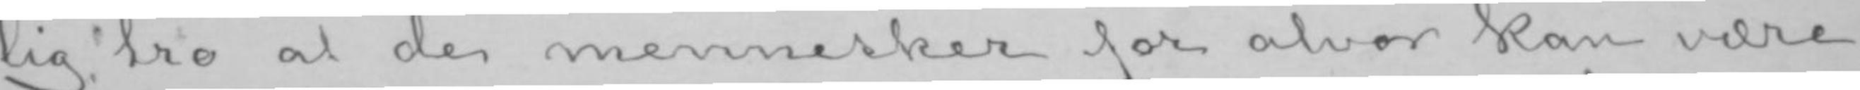lig tro at de mennesker for alvor kan være
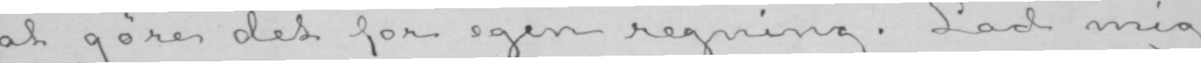at gøre det for egen regning. Lad mig
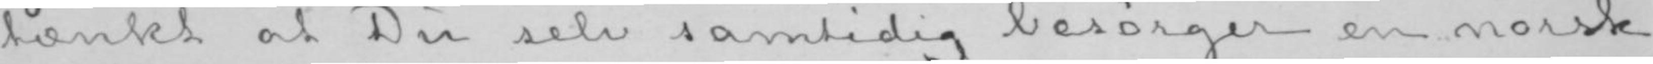tænkt at Du selv samtidig besørger en norsk
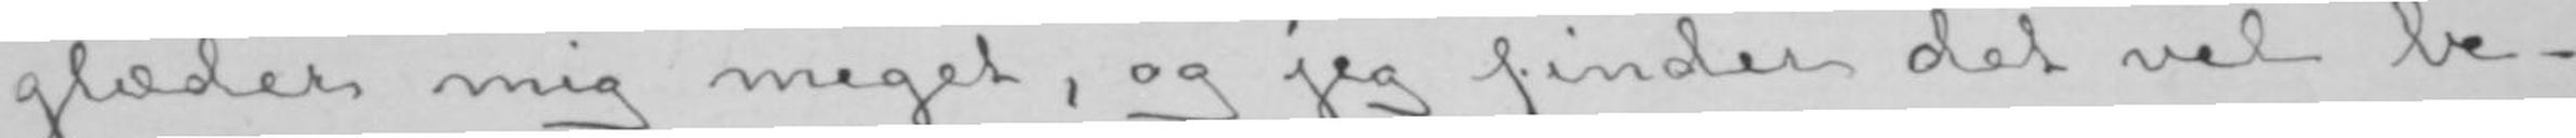glæder mig meget, og jeg finder det vel be-
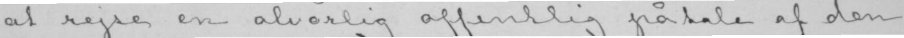at rejse en alvorlig offentlig påtale af den
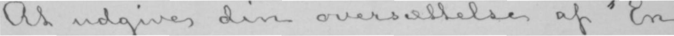At udgive din oversættelse af «En
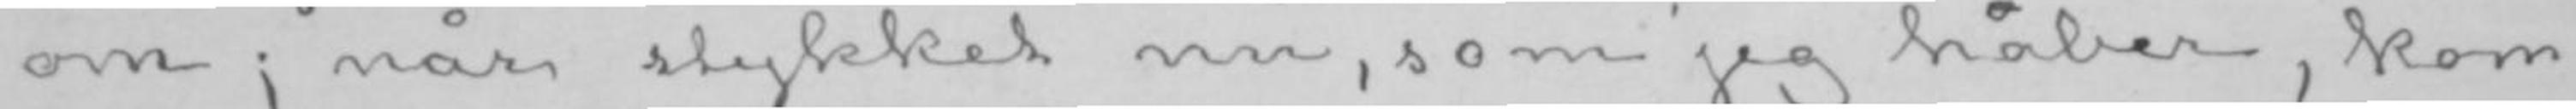om; når stykket nu, som jeg håber, kom-
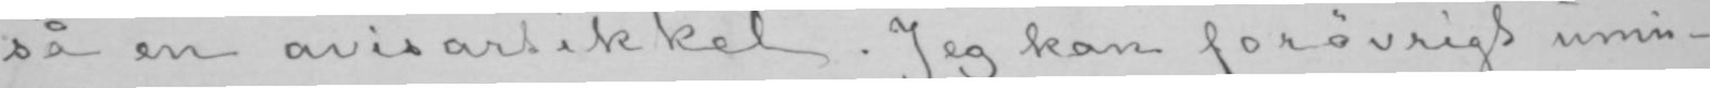så en avisartikkel. Jeg kan forøvrigt umu-
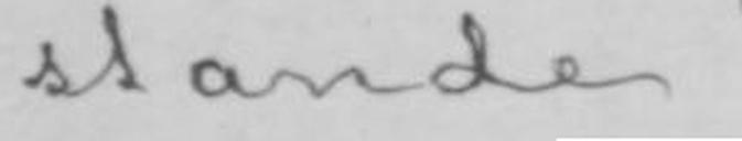stande!
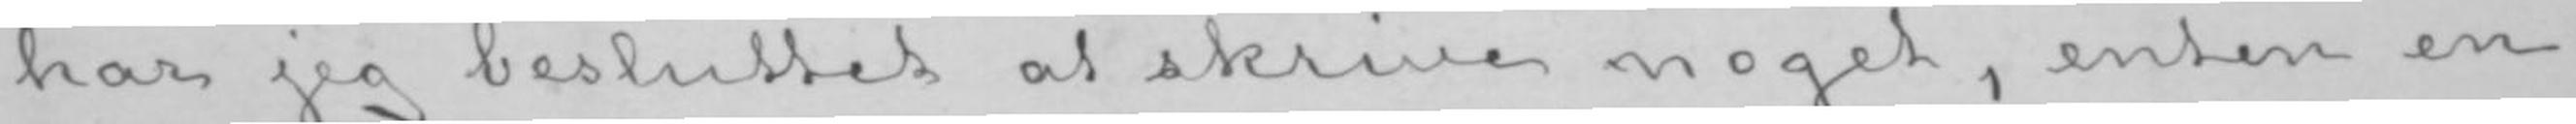har jeg besluttet at skrive noget, enten en
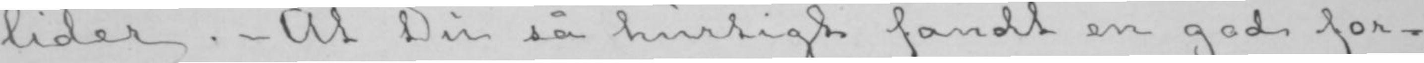lider.- At Du så hurtigt fandt en god for-
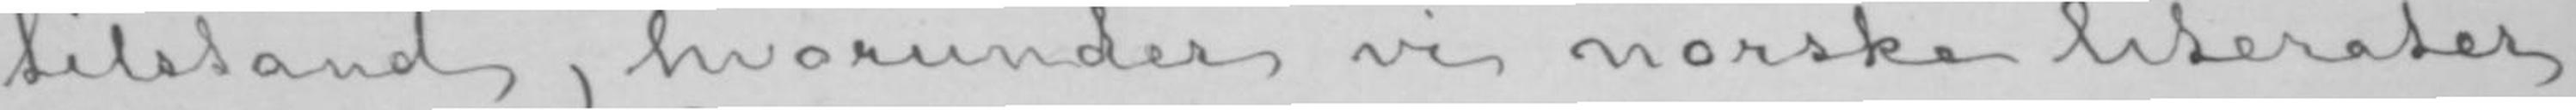tilstand, hvorunder vi norske literater
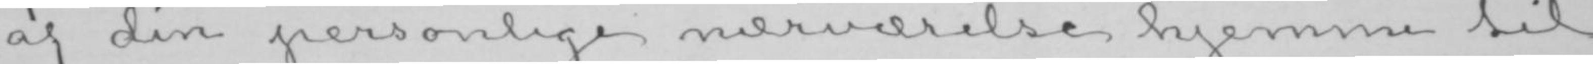af din personlige nærværelse hjemme til
